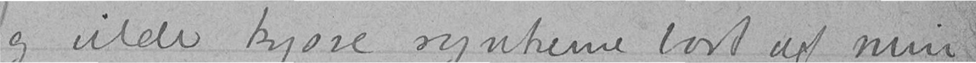og vilde kysse rynkerne bort af min
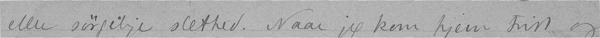eller sørgelige slethed. Naar jeg kom hjem trist og
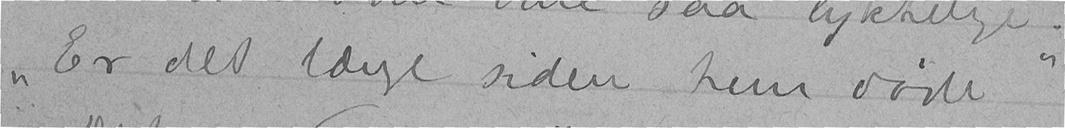"Er det længe siden hun døde"
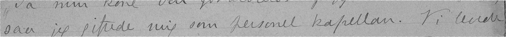saa jeg giftede mig som personel kapellan. Vi levede
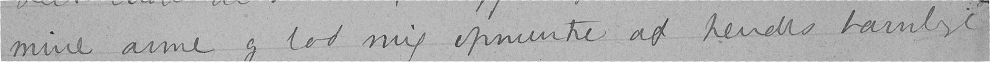mine arme og lod mig opmuntre af hendes barnlige
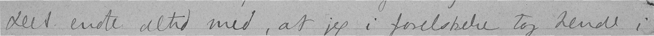Det endte altid med, at jeg i forelskelse tog hende i
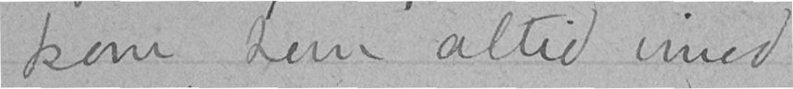kom hun altid imod
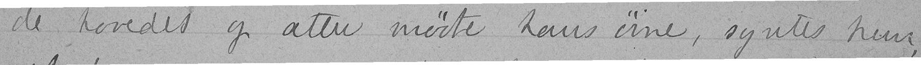de hovedet og atter mødte hans øine, syntes hun
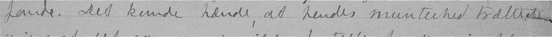pande. Det kunde hænde, at hendes munterhed trættede
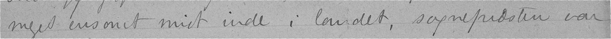meget ensomt midt inde i landet, sognepræsten var
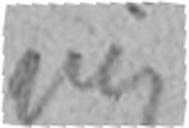mig
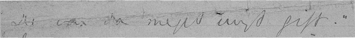"De var da meget ungt gift."
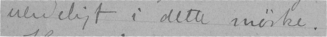uendeligt i dette mørke.
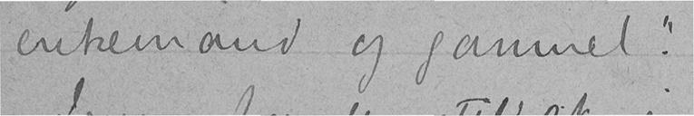enkemand og gammel."
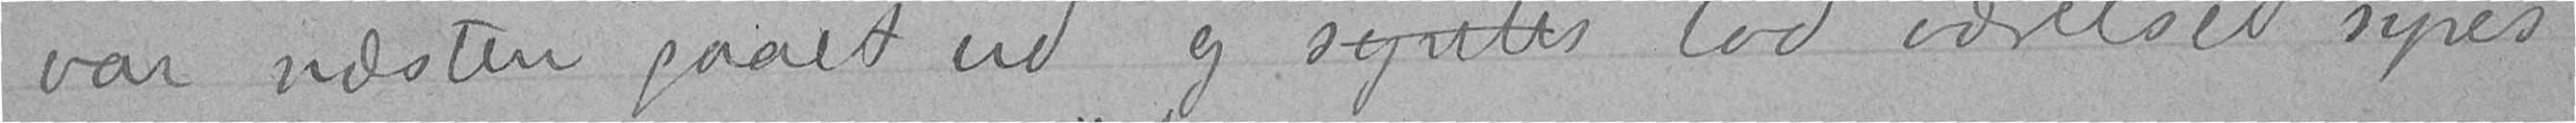var næsten gaaet ud og syntes lod værelset synes
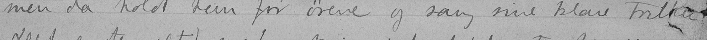men da holdt hun for ørene og sang sine klare triller.
